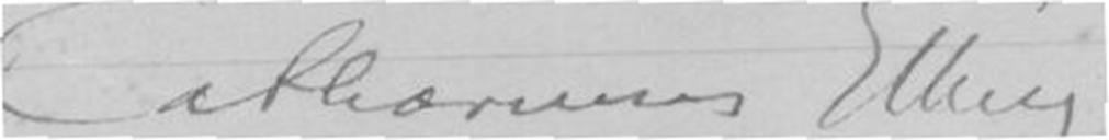Catharinus Elling
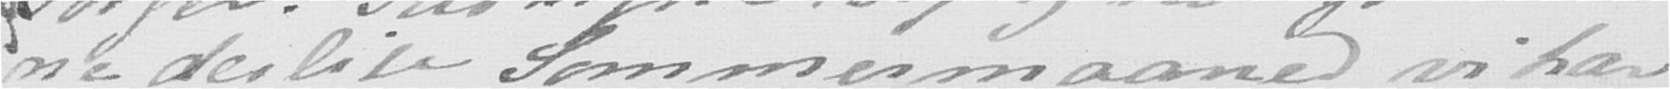ne deilige Sommermaaned vi har
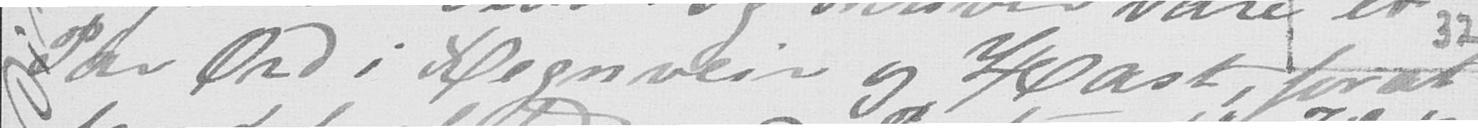Par Ord i Regnveir og Hast, forat
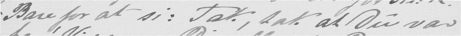Bare for at si: Tak, tak at Du var
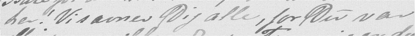her! Vi savner Dig alle, for Du var
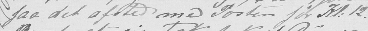faa det afsted med Posten for Kl: 12.
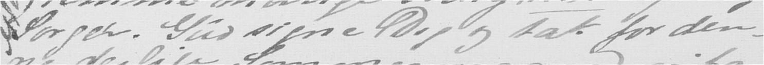Sorger. Gud signe Dig og tak for den-
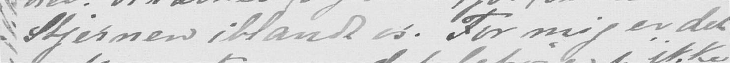Stjernen iblandt os. For mig er det
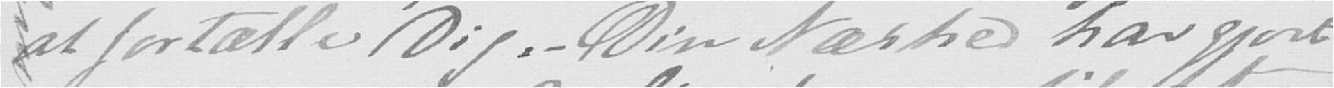at fortælle Dig. Din Nærhed har gjort
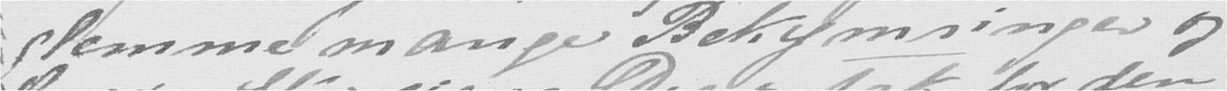glemme mange Bekymringer og
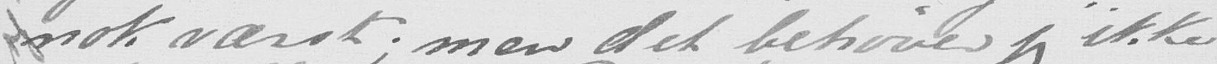nok værst; men det behøver jeg ikke
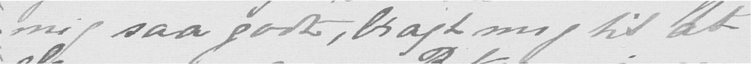mig saa godt, bragt mig til at
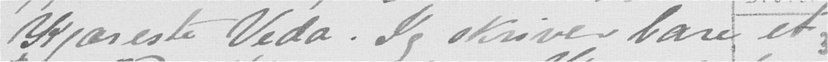Kjæreste Veda. Jeg skriver bare et-
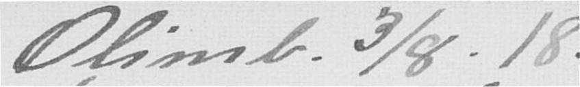Olimb. 3/8. 18.
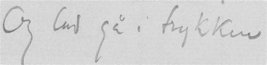Og lad gå i trykken
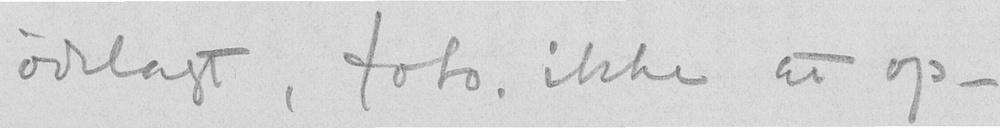ødelagt, foto. ikke at op-
